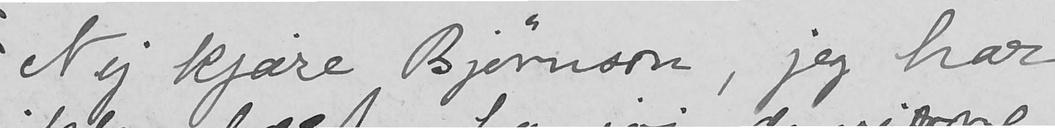Nej kjære Bjørnson, jeg har
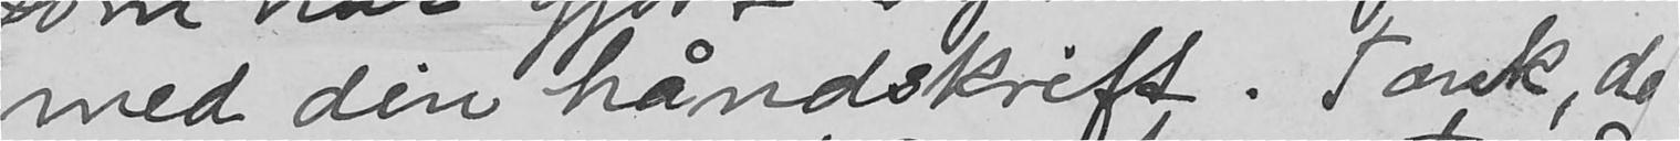med den håndskrift. Tænk, de
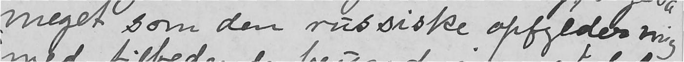meget som den russiske opfylder mig
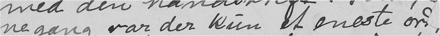negang var der kun et eneste ord
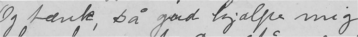Og tænk, så gud hjælpe mig
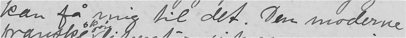kan få mig til det. Den moderne
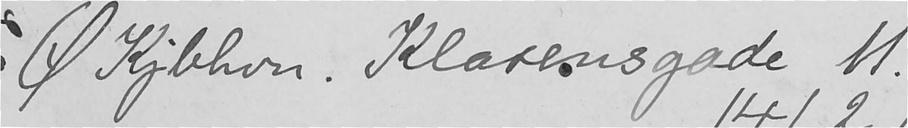Ø Kjbhvn. Klasensgade 11.
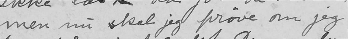men nu skal jeg prøve om jeg
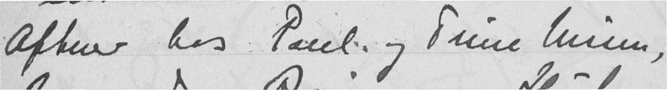Aftener hos Paul og Trine Nissen,
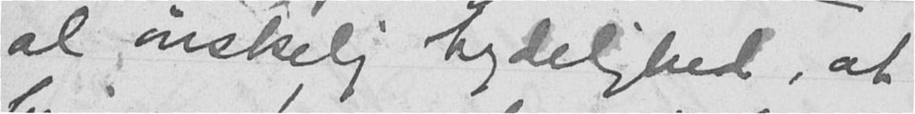al ønskelig tydelighed, at
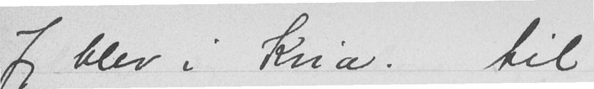Jeg blev i Kria. til
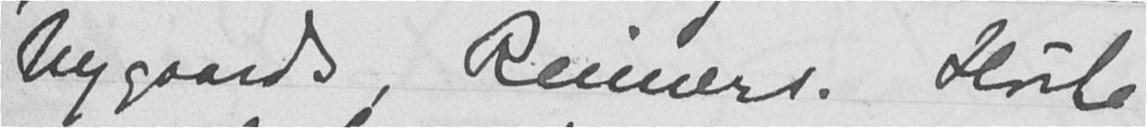Nygaards, Reimers. Hørte
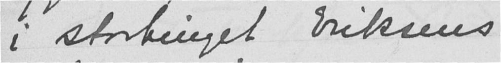i stortinget Eriksens
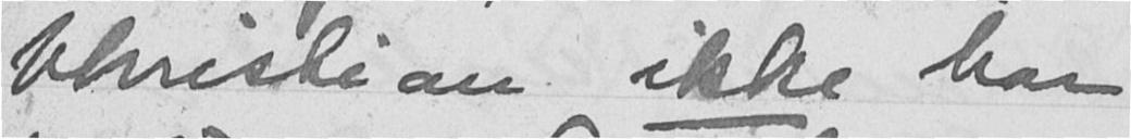Christian ikke har
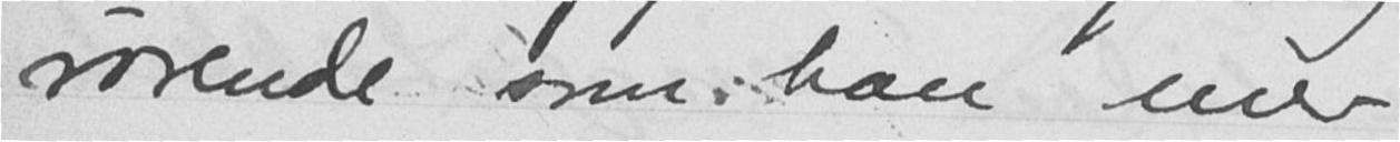rørende som han mer
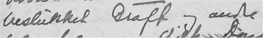bestukket Graff og andre
